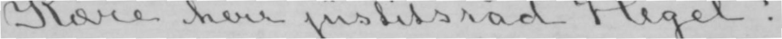Kære herr justitsråd Hegel!
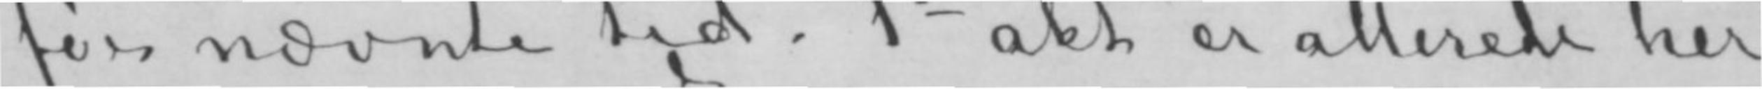før nævnte tid. 1ste akt er allerede her
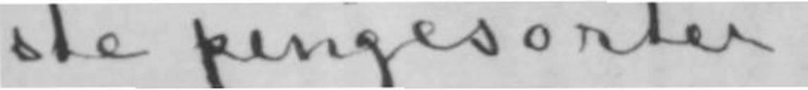ste pengesorter.
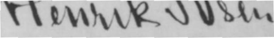Henrik Ibsen.
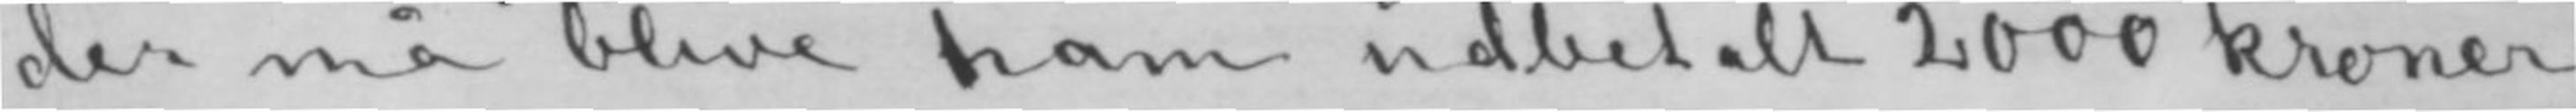der må blive ham udbetalt 2000 kroner
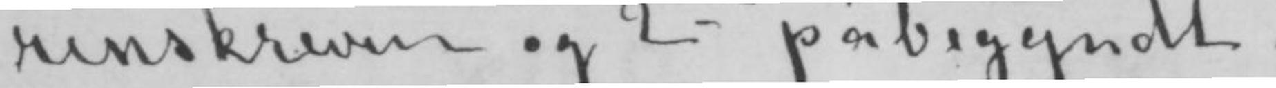renskreven og 2den påbegyndt.
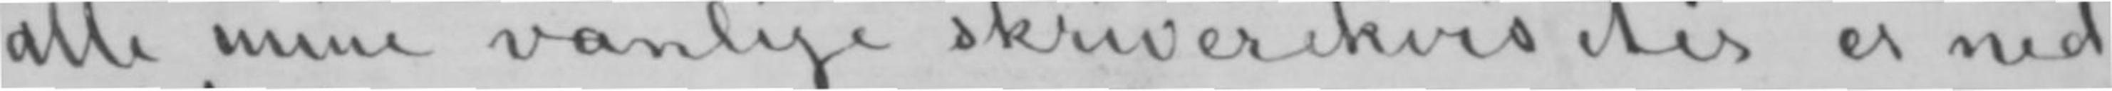alle mine vanlige skriverekvisiter er ned-
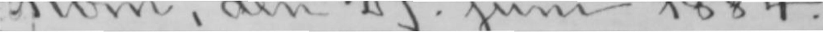Rom, den 29. juni 1884.
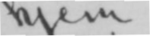hjem.
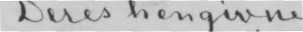Deres hengivne
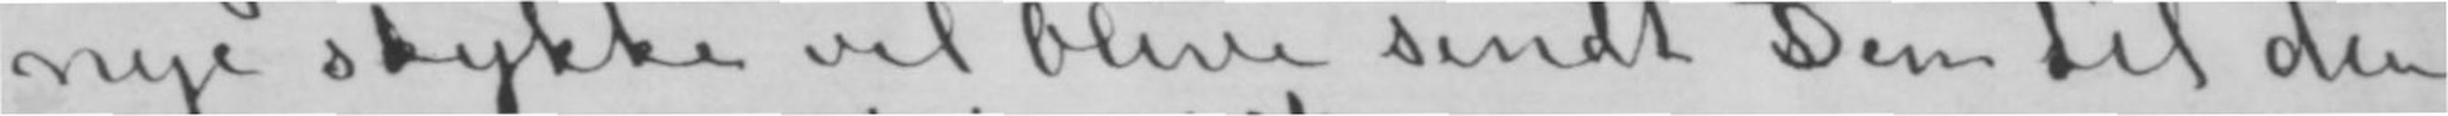nye stykke vil blive sendt Dem til den
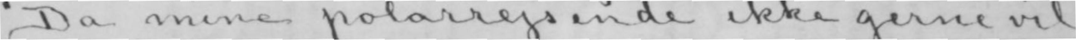Da mine polarrejsende ikke gerne vil
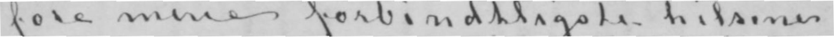føre mine forbindtligste hilsener.
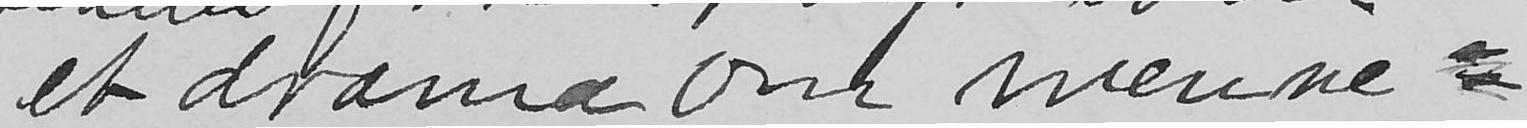et drama om menne-
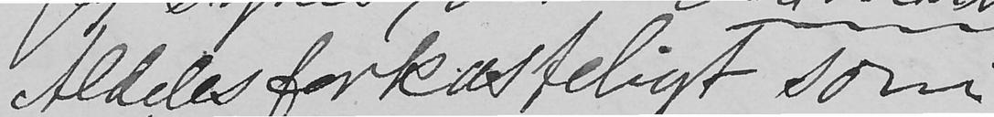Aldeles forkasteligt som
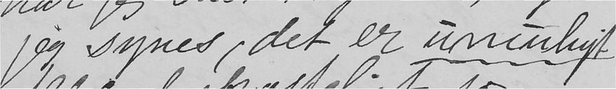jeg synes, det er umuligt
